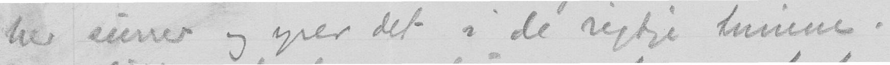her suser og yrer det i de rigtige timene.
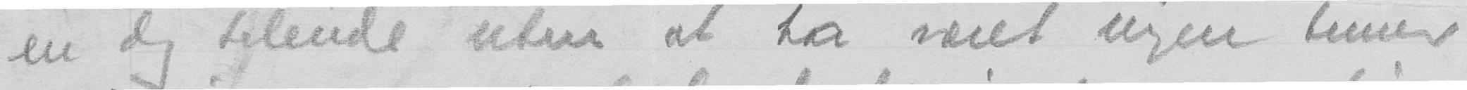en dag tilende uten at ha været nogen timer
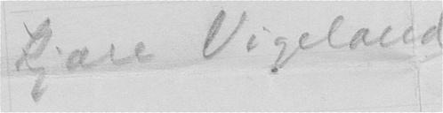Kjære Vigeland
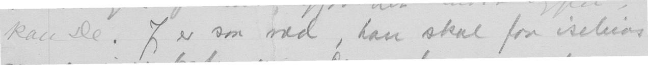kan De. Jeg er saa ræd, han skal faa iselnos
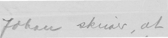Johan skriver, at
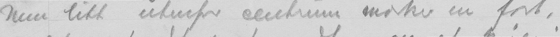Men litt utenfor centrum mærker en fort,
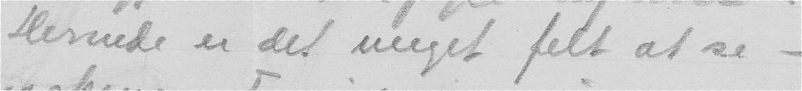Hernede er det meget felt at se -
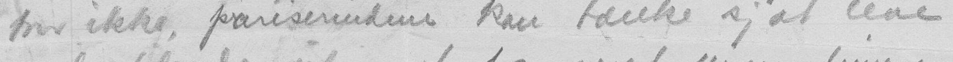tror ikke, pariserindene kan tænke sig at leve
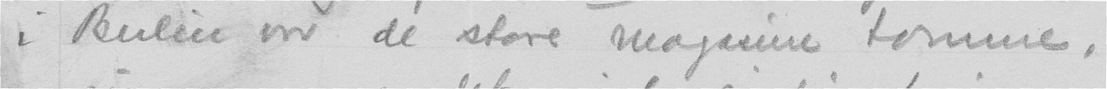i Berlin er de store magasine tomme,
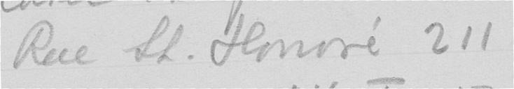Rue St. Honore 211
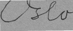Oslo
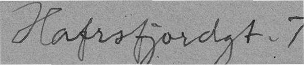Hafrsfjordgt. 7
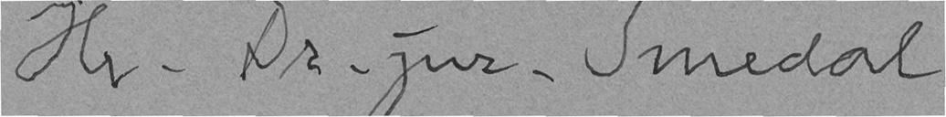Hr. Dr. jur. Smedal
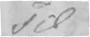Til
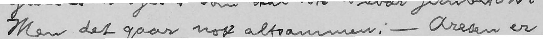Men det gaar nok altsammen; - Aresen er
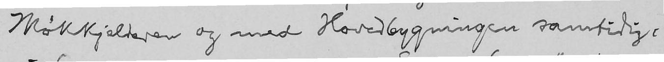Møkkjelderen og med Hovedbygningen samtidig,
